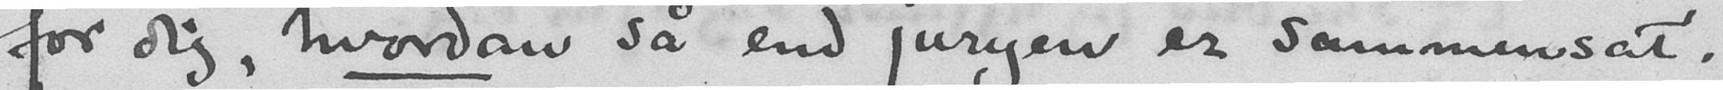for dig, hvordan så end juryen er sammensat.
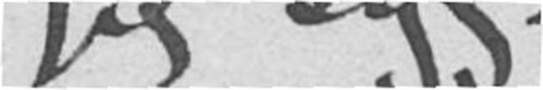jeg syg.
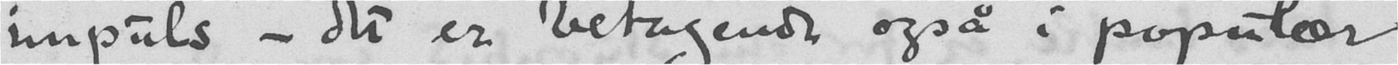impuls - det er betagende også i populær
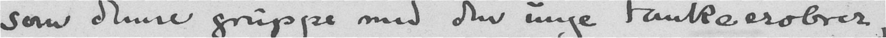som denne gruppe med den unge tankeerobrer,
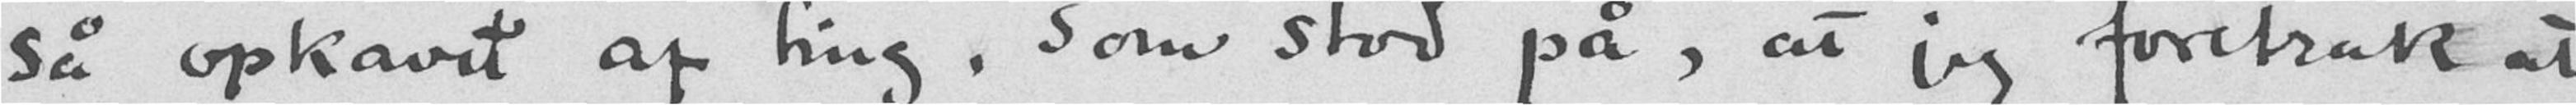så opkavet af ting, som stod på, at jeg foretrak at
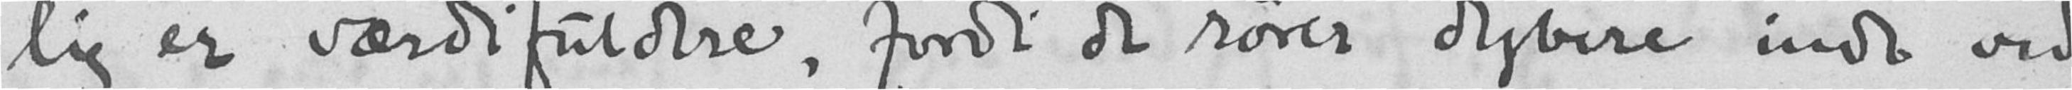lig er værdifuldere, fordi de rører dybere inde ved
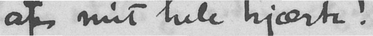af mit hele hjærte!
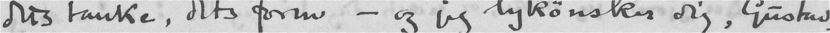dets tanke, dets form - og jeg lykønsker dig, Gustav,
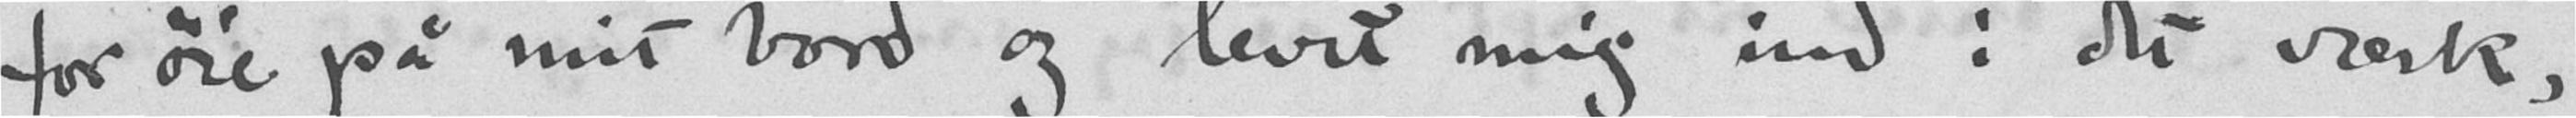for øie på mit bord og levet mig ind i det værk,
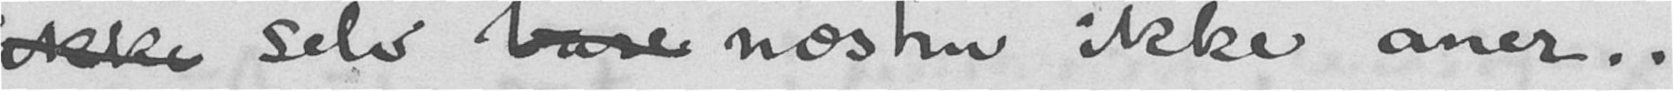ikke selv bare næsten ikke aner..
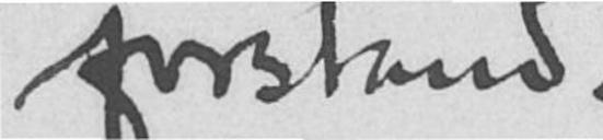forstand.
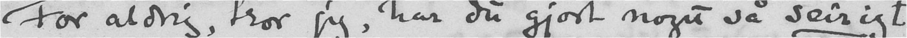For aldrig, tror jeg, har du gjort noget så seirigt
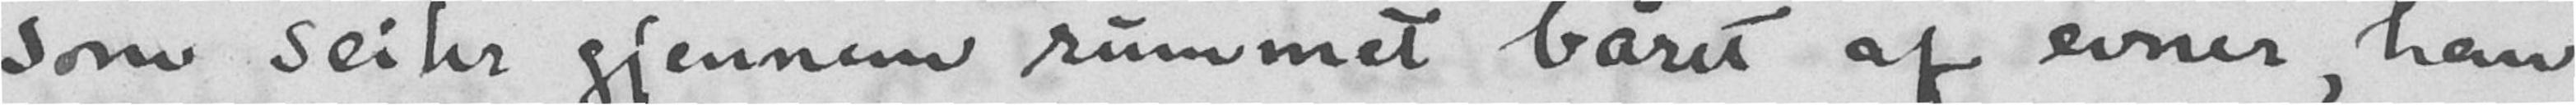som seiler gjennem rummet båret af evner, han
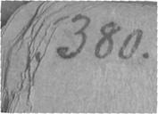380.
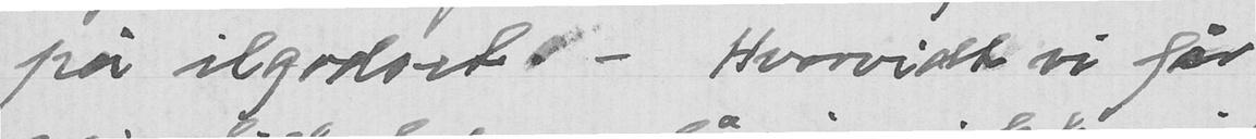på ilgodset. - Hvorvidt vi får
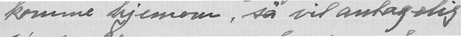komme hjemom, så vil antagelig
